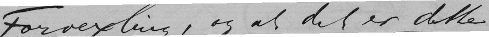Forvexling, og at det er dette
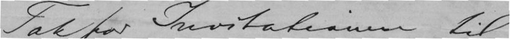Tak for Invitationen til
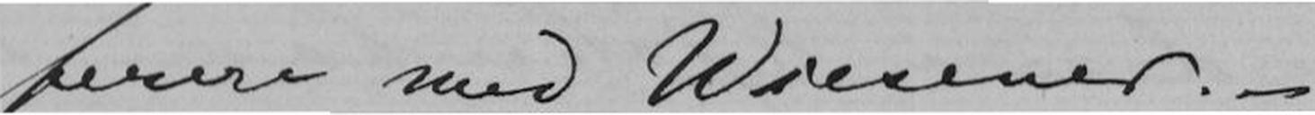ferere med Wiesener.-
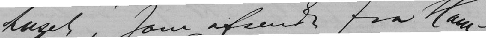huset, som afsendt fra Ham-
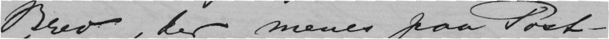Brev, der menes paa Post-
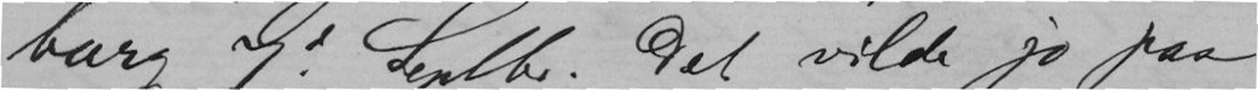burg 7de Septbr. Det vilde jo pas-
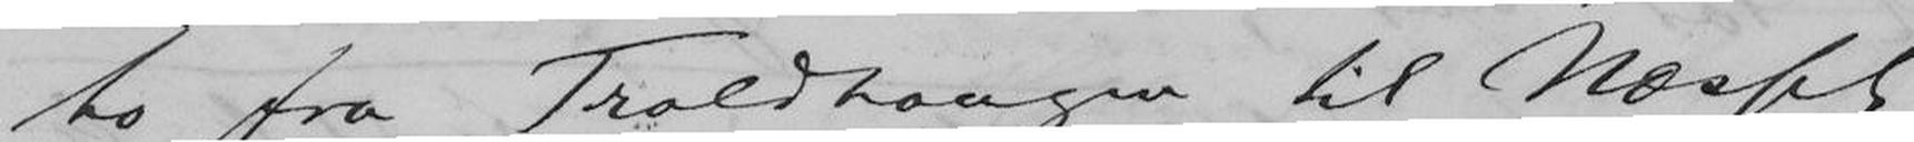ho fra Troldhaugen til Næsset
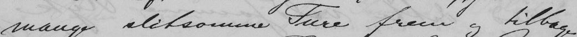mange slitsomme Ture frem og tilbage
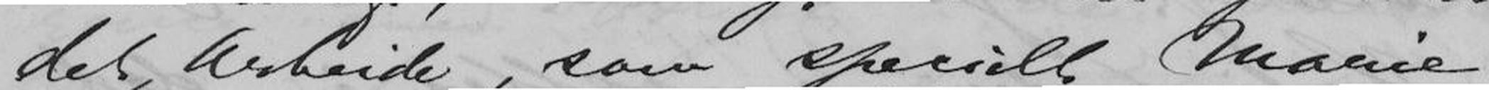det Arbeide, som specielt Marie
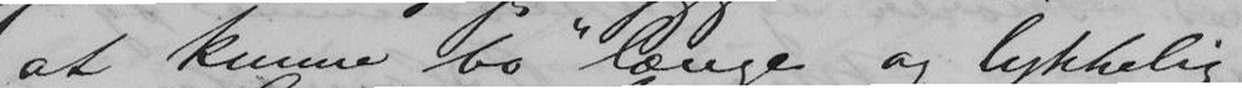at kunne bo "længe og lykkelig"
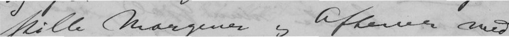stille Morgener og Aftener med
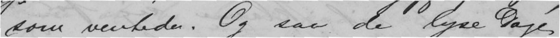som ventede. Og saa de lyse Dage
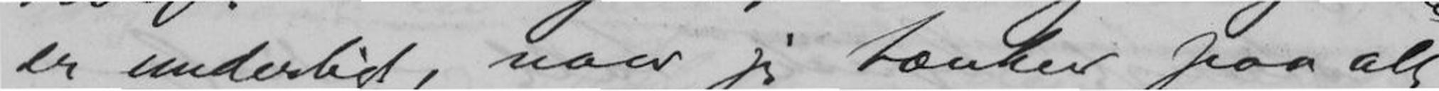er underligt, naar jeg tænker paa alt
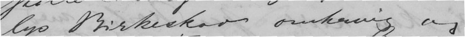lys Birkeskov omkring og
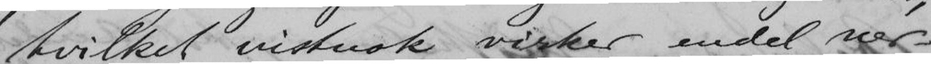hvilket vistnok virker endel ner-
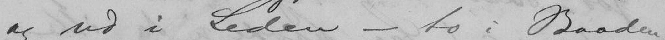og ud i Leden - to i Baaden -
